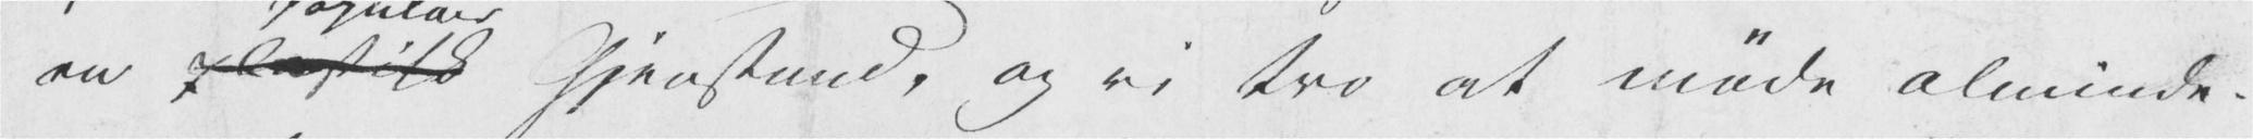en plastisk Gjenstand, og vi tro at møde alminde-
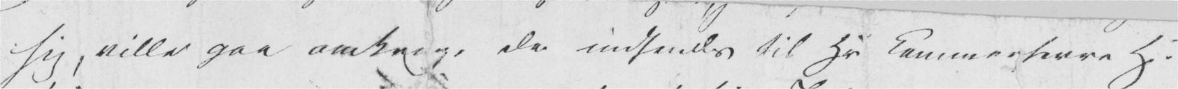sig, ville gaa omkring, de indsendes til Hr Kammerherre H.
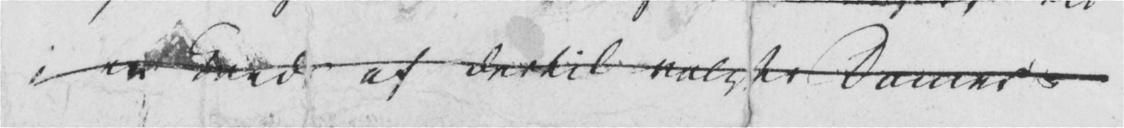i en Kreds af dertil valgte Damer.
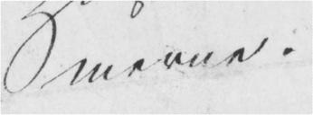merne.
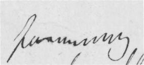<...>
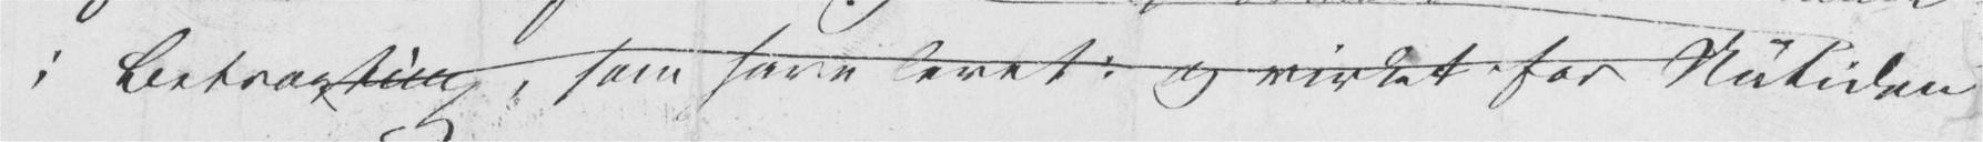i Betragtning, som have levet i og virket for Nutiden.
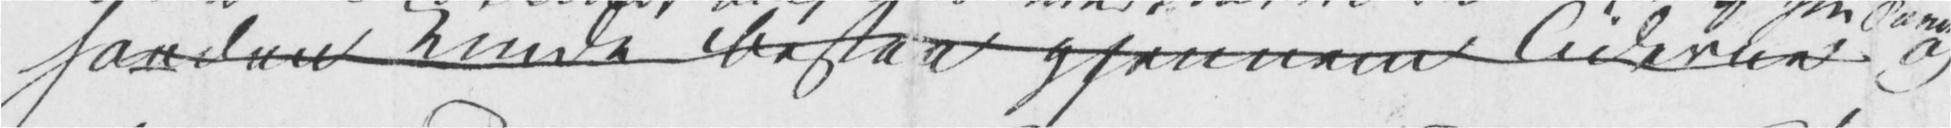saadan kunde bestaa gjennem Tiderne og
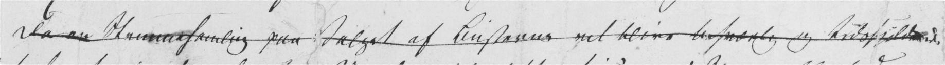Da en Stemmesamling paa Valget af Busterne vil blive besværlig og tidsspildende
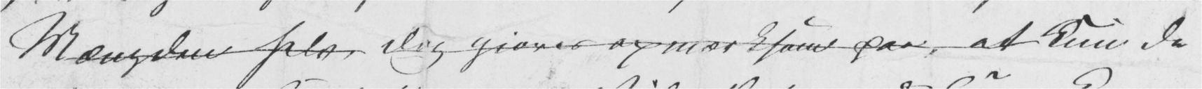Mængden selv, dog gjøres opmærksom paa, at kun de
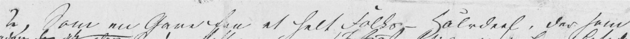2, Som en Gave fra et helt Folks – Halvdeel, der som
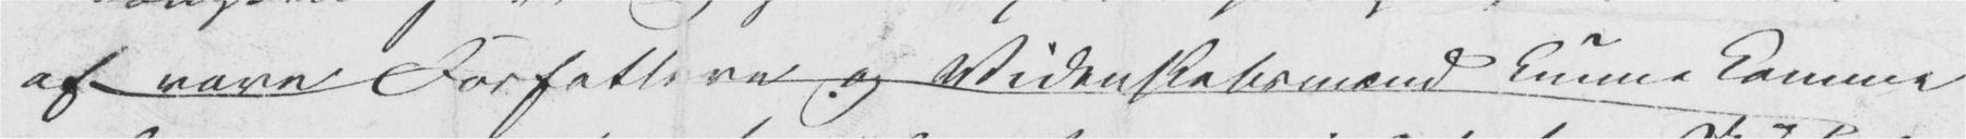af vore Forfattere og Videnskabsmænd kunne komme
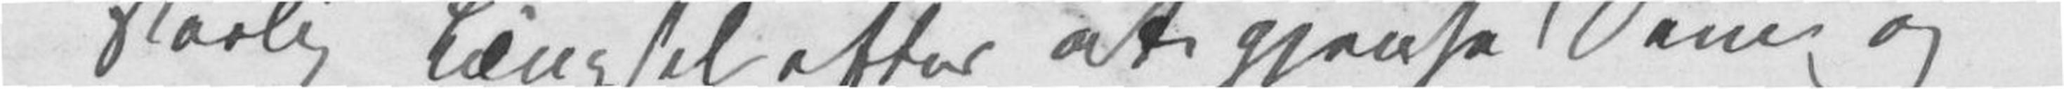ståelig Længsel efter at gjense Dem og
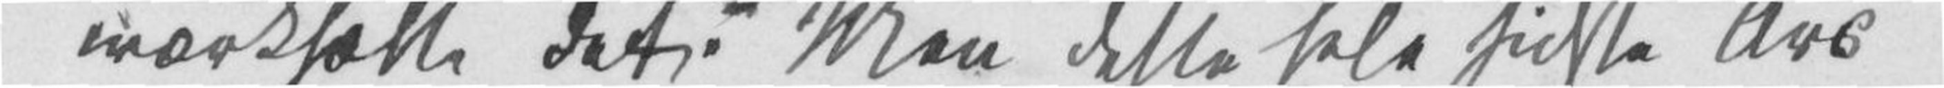iværksætte det! Men dette hele sidste Års
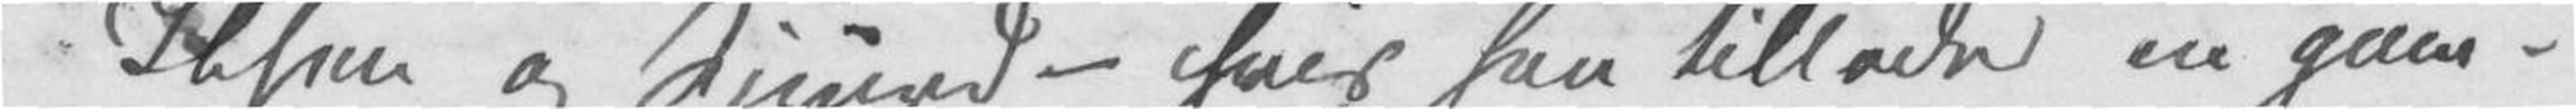Ibsen og Sigurd – hvis han tillader en gam¬
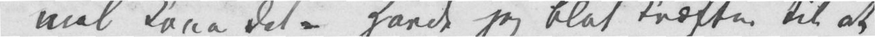mel Kone det. Havde jeg blot Kræfter til at
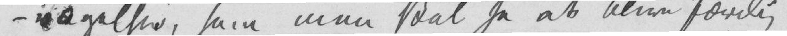vægelser, som man skal se at blive færdig
